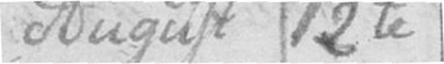August 12te
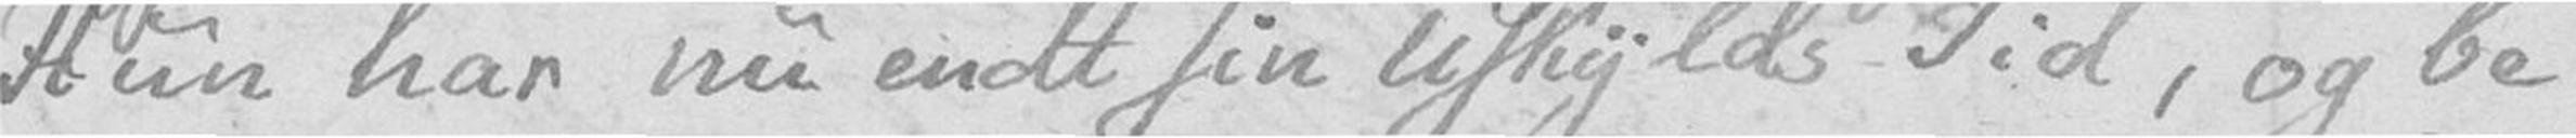Hun har nu endt sin Uskylds Tid, og be
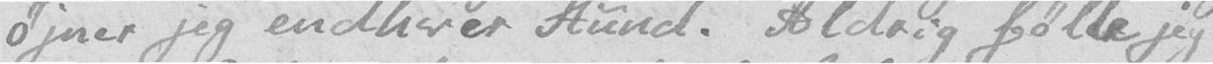øjner jeg endhver Stund. Aldrig følte jeg
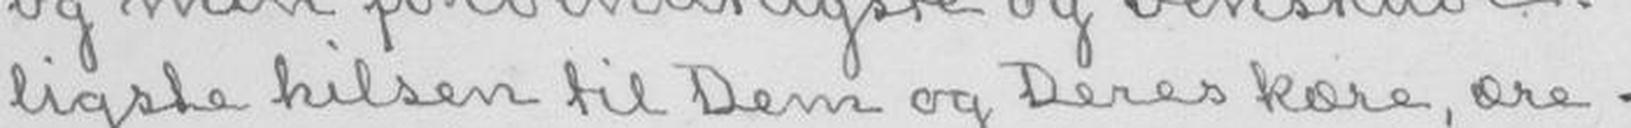ligste hilsen til Dem og Deres kære, ære-
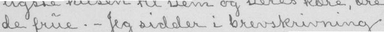de frue. - Jeg sidder i brevskrivning
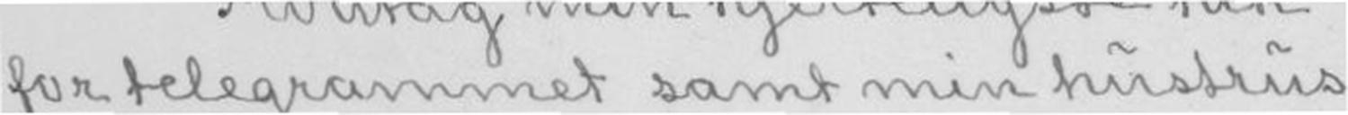for telegrammet samt min hustrus
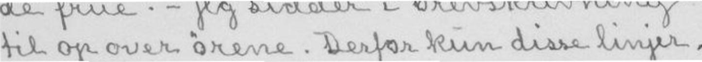til op over ørene. Derfor kun disse linjer.
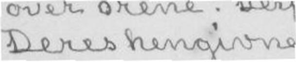Deres hengivne
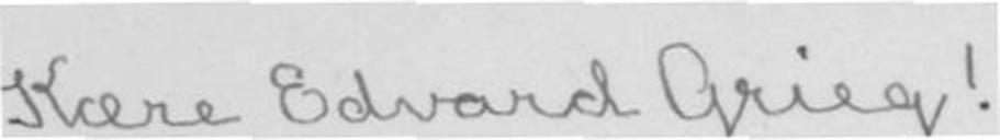Kære Edvard Grieg!
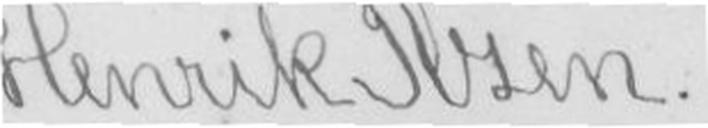Henrik Ibsen
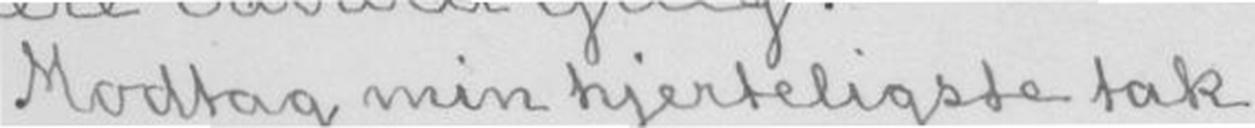Modtag min hjerteligste tak
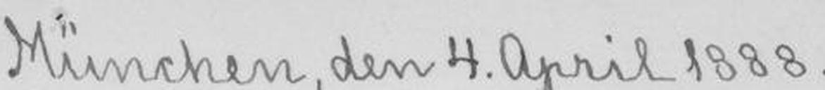München, den 4. April 1888.
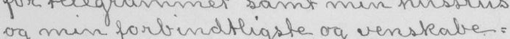og min forbindtligste og venskabe-
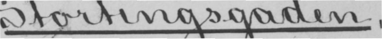Stortingsgaden,
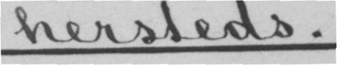hersteds.
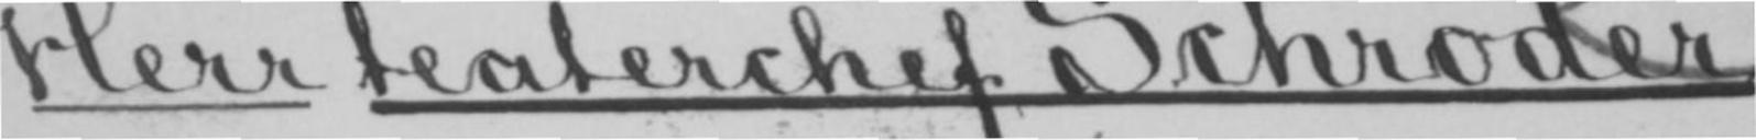Herr teaterchef Schrøder,
